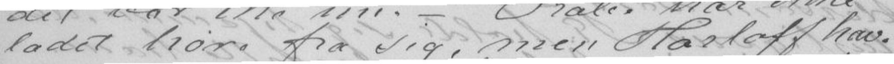ladet høre fra sig, men Harloff hav-
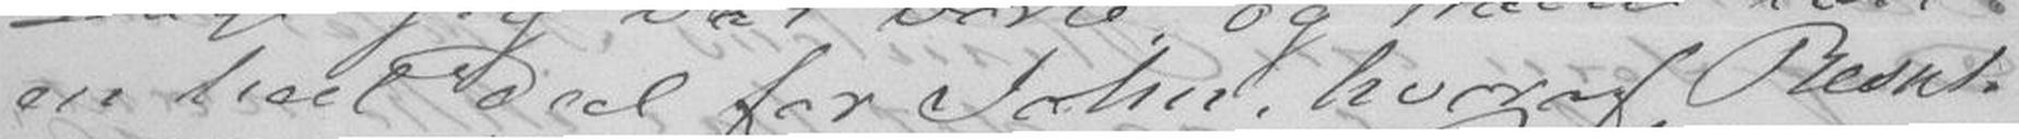en heel Deel for John, hvoraf Resul-
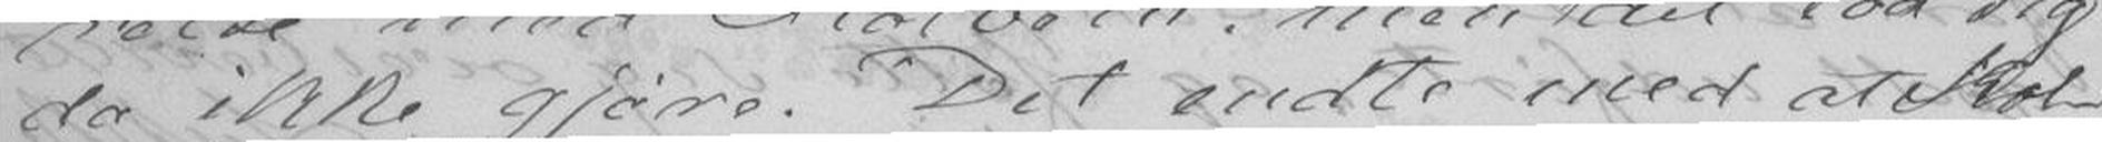da ikke gjøre. Det endte med at Kol-
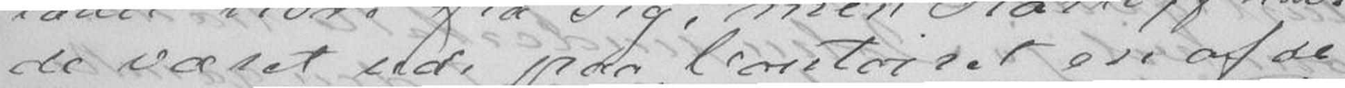de været ud paa Contoiret en af de
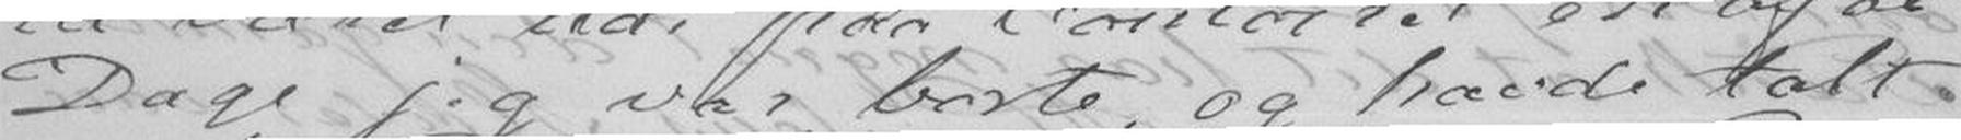Dage jeg var borte, og havde talt
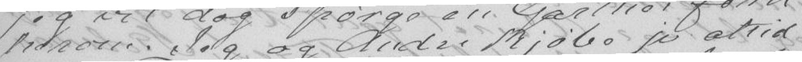herom. Jeg og Andre kjøbe jo altid
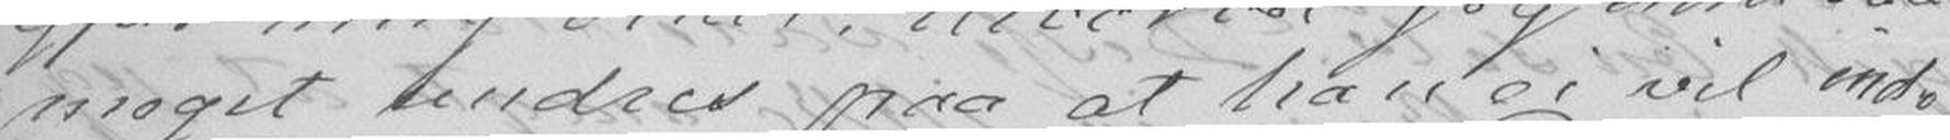meget undres paa at han ei vil ind-
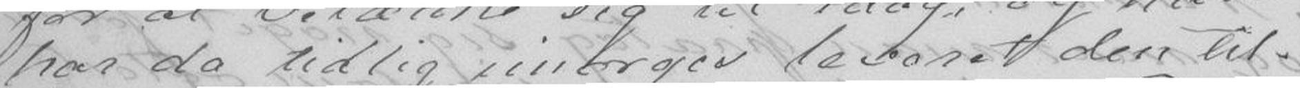har da tidlig imorgen leveret den til-
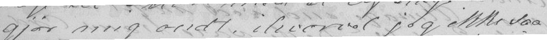gjør mig ondt, ihvorvel jeg ikke saa
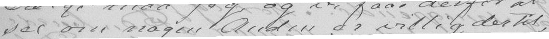see om nogen Anden er villig dertil,
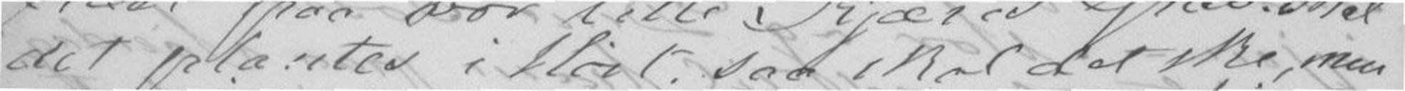der plantes i Høst, saa skal det ske, men
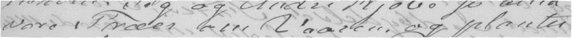vore Træer om Vaaren, og planter
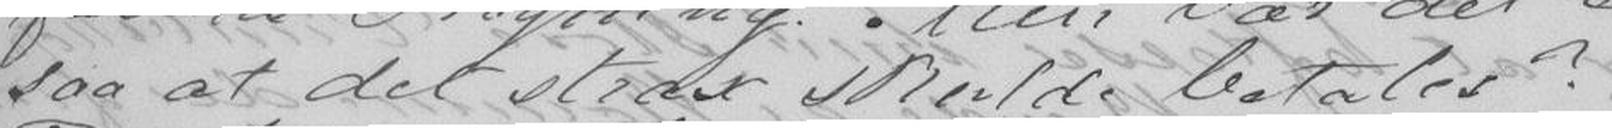saa at det strax skulde betales?
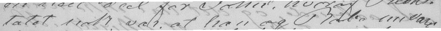tatet nok var at han og Rabe nu var
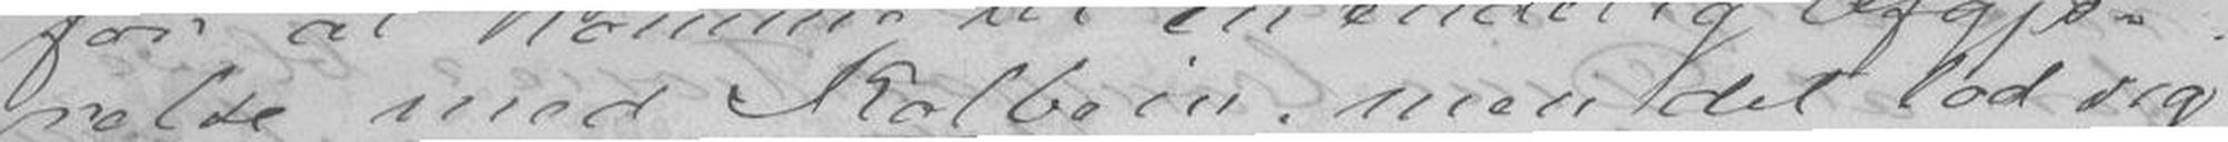relse med Kolbein, men det lod sig
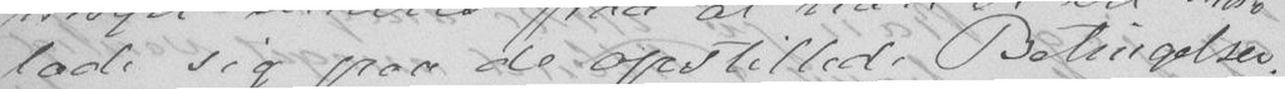lade sig paa de opstilled Betingelser.
